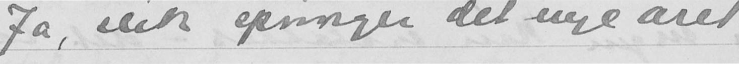Ja, slik springer det nye året
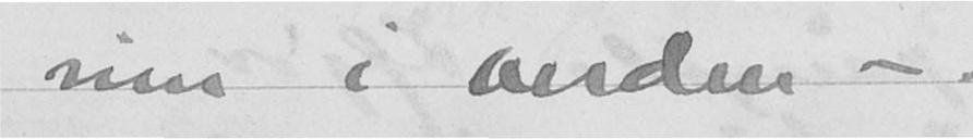inn i verden -.
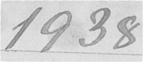1938
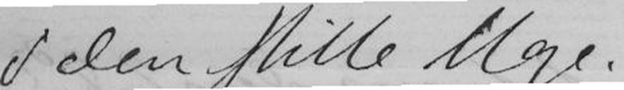i den stille Uge.
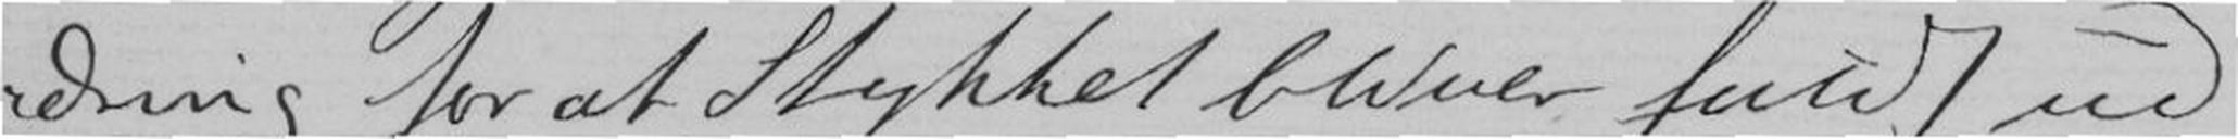dring for at Stykket bliver fuldt ud
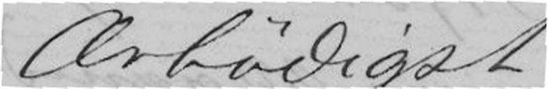Ærbødigst
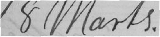18 Marts.
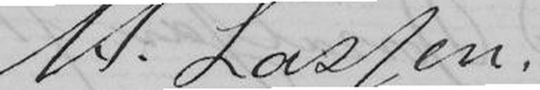M. Lassen.
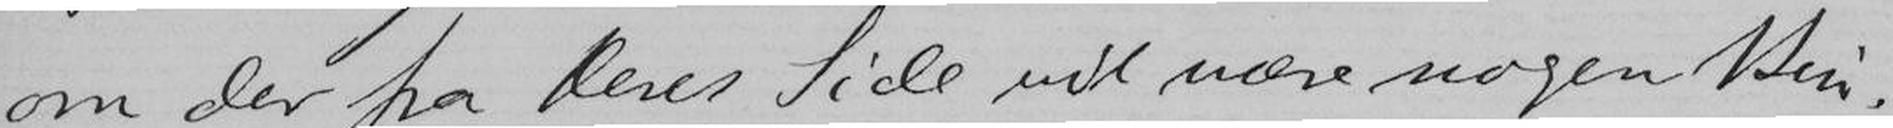om der fra Deres Side vil være nogen Hin-
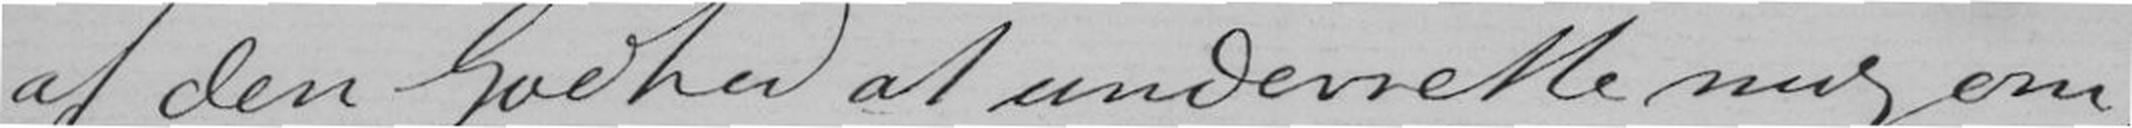af den Godhed at underrette mig om,
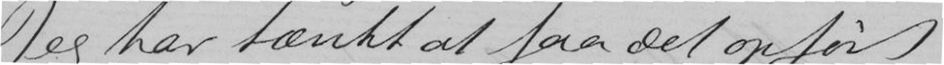Jeg har tænkt at faa det opført
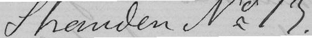Shanden No
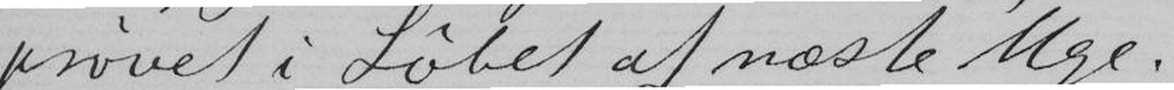prøvet i Løbet af næste Uge.
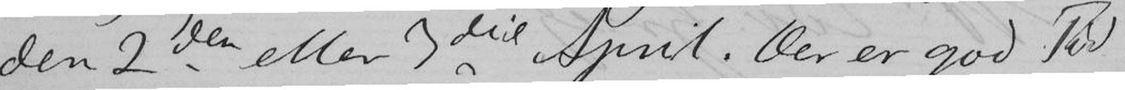den 2den eller 3die April. Der er god Tid
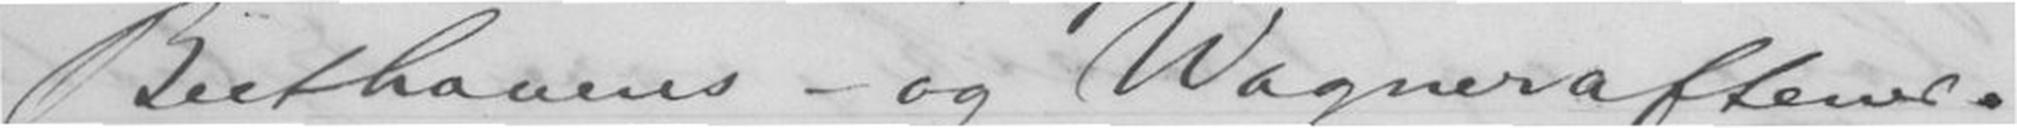Beethovens- og Wagneraftener.
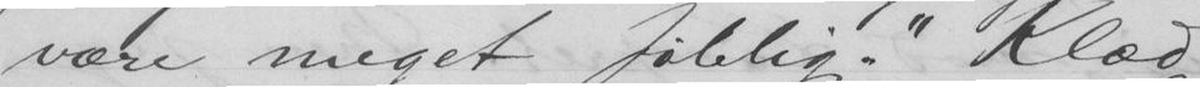være meget følelig. "Klæd
